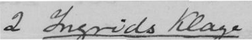2. Ingrids Klage
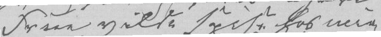Frue vilde spise hos mig
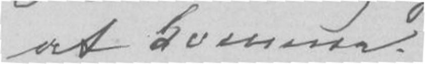at komme.
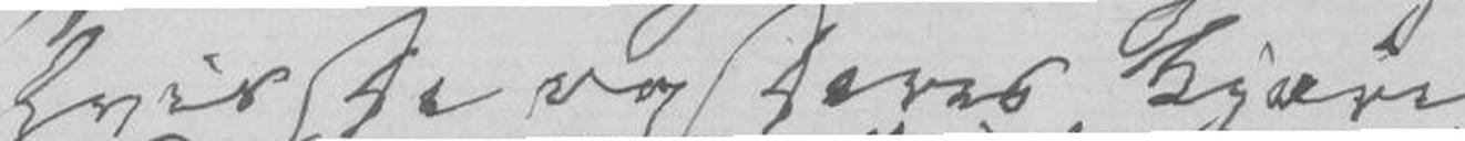hvis De og Deres kjære
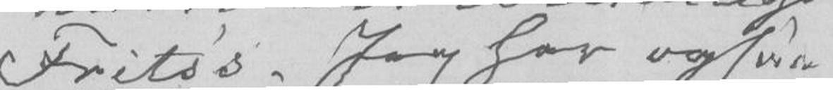Frits.s. Jeg har ogsaa
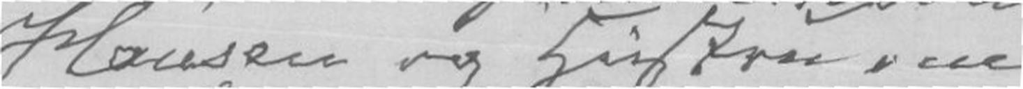Hansen og Hustru om
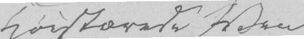Høistærede Ven
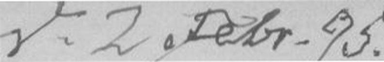d. 2 Febr. 95
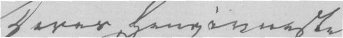Deres hengivende
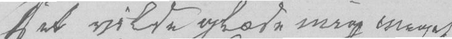Det vilde glæde mig meget
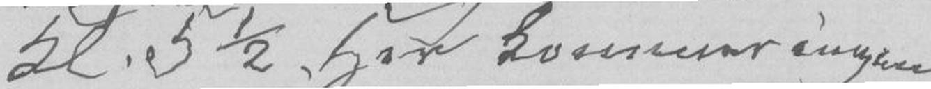Kl. 5 1/2. Her kommer ingen,
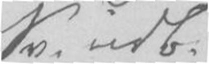Sv. udb.
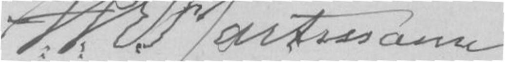J.P.E.Hartmann
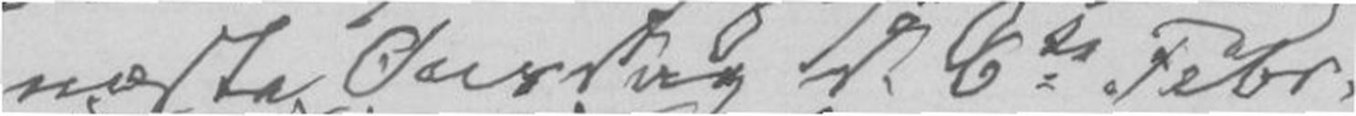næste Onsdag d. 6te Febr.
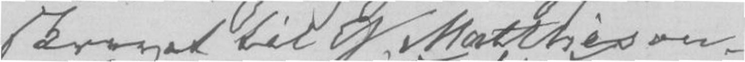skrevet til G. Matthison-
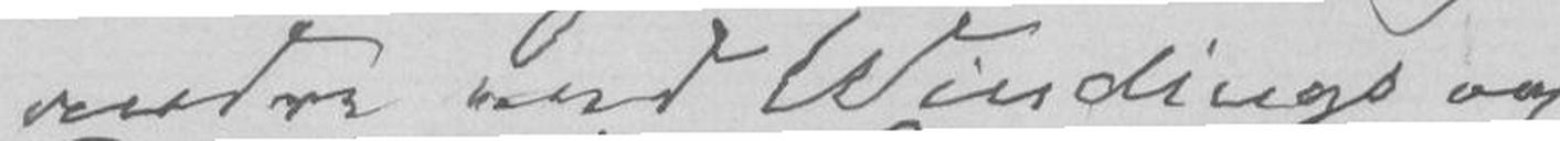andre end Windings og
